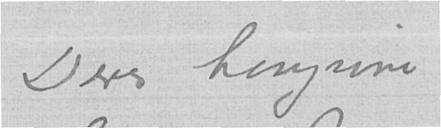Deres hengivne
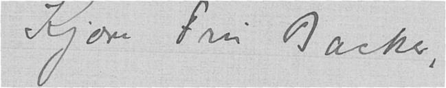Kjære Fru Backer,
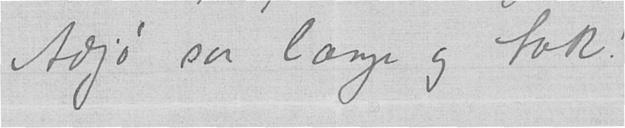Adjø saa længe og tak!
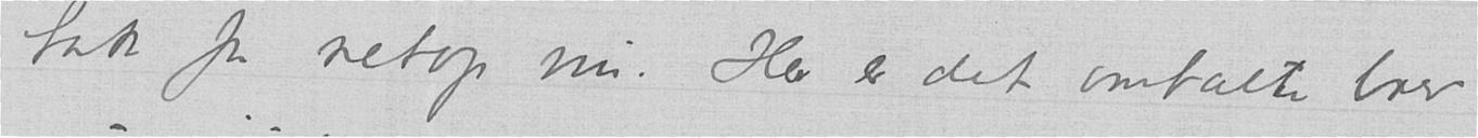tak for netop nu. Her er det omtalte brev
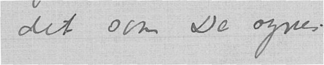det som De synes
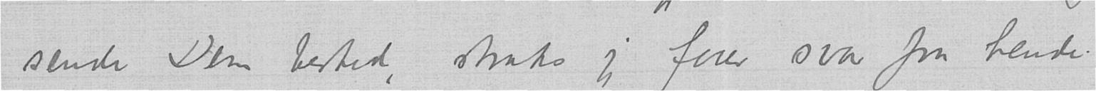sende Dem besked, straks jeg faar svar fra hende.
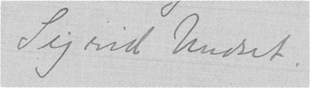Sigrid Undset.
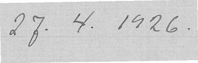27.4.1926.
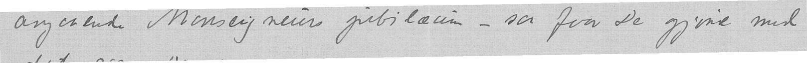angaaende Monseigneurs jubilæum – saa faar De gjøre med
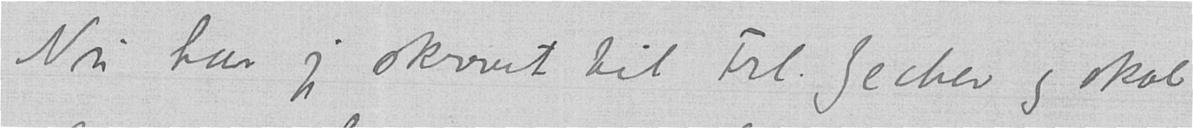Nu har jeg skrevet til Frk. Zecher og skal
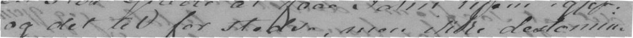og det til for stedse, men ikke destomin-
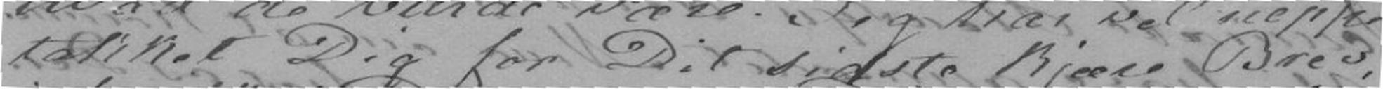takket Dig for Dit sidste kjære Brev,
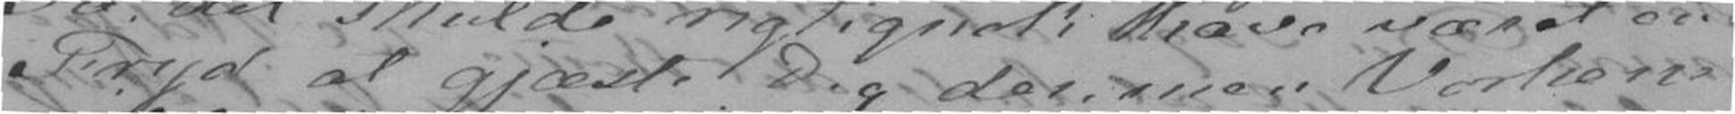Fryd at gjæste Dig der, men Vorherre
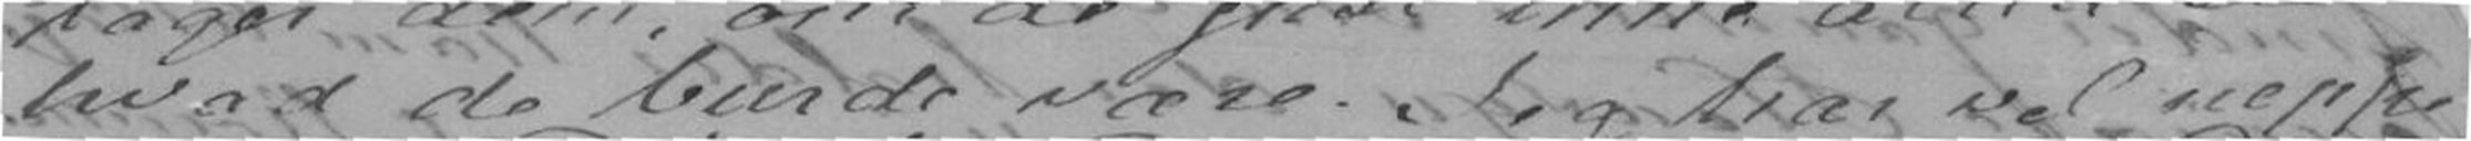hvad de burde være. Jeg har vel neppe
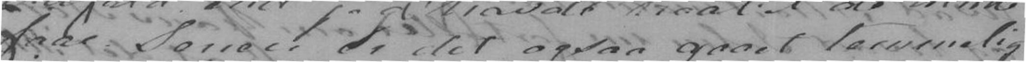faae. Senere er det ogsaa gaaet temmelig
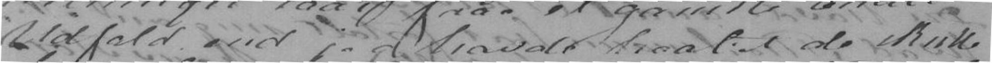Udfald end jeg havde haabet de skulle
